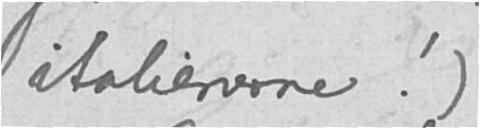italienerne!)
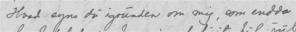Hvad syns du igrunden om mig, som endda
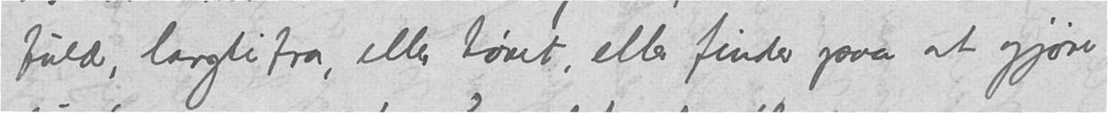fuld, langtifra, eller tøvet, eller finder paa at gjøre
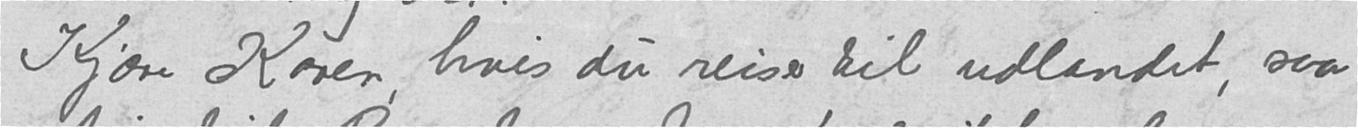Kjære Karen hvis du reiser til udlandet, saa
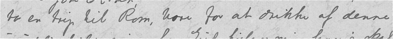ta en trip til Rom, bare for at drikke af denne
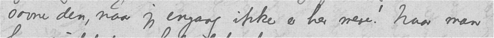savne den, naar jeg engang ikke er her mere! Naar man
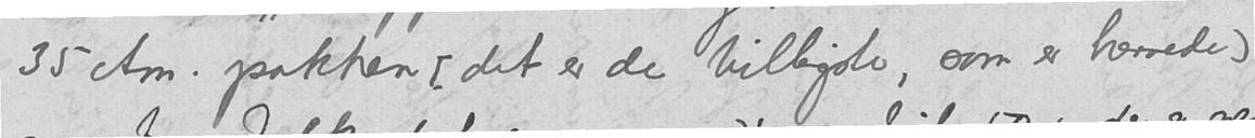35 ctm. pakken (det er de billigste, som er hernede)
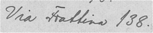Via Frattina 138.
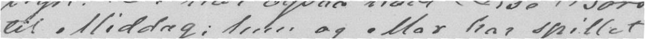til Middag; hun og Max har spillet
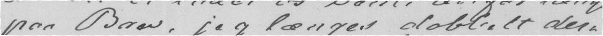paa Brev, jeg længes dobbelt der-
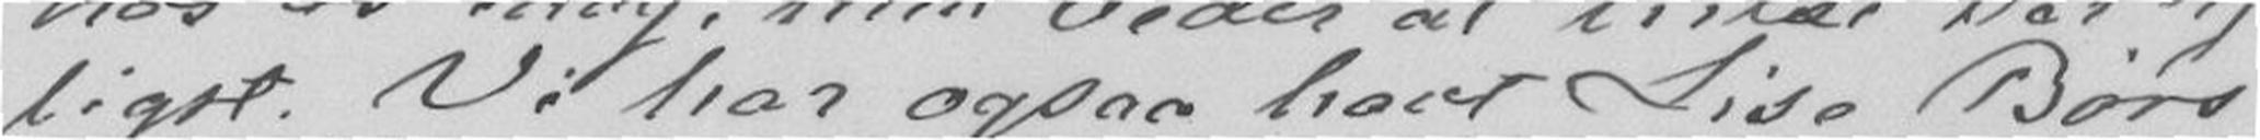ligst. Vi har ogsaa havt Lise Børs
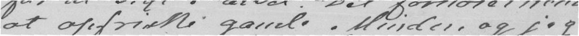at opfriske gamle Minder, og jeg
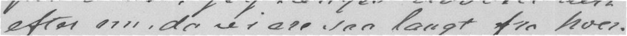efter nu da vi ere saa langt fra hver-
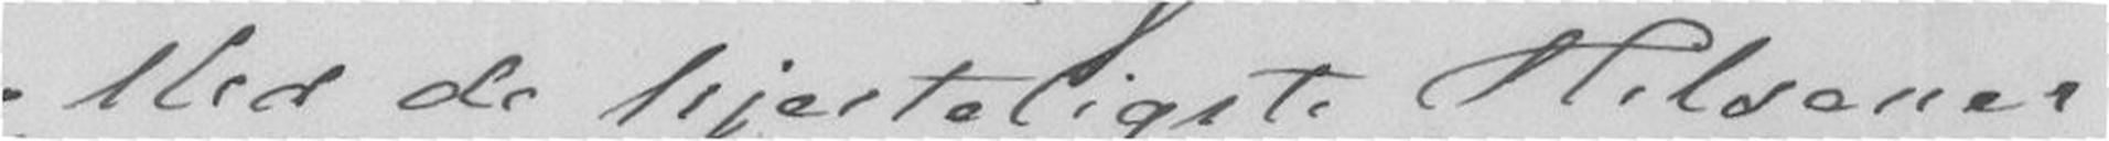Med de hjerteligste Hilsener
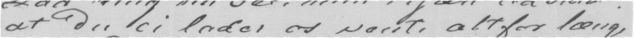at Du ei lader os vente altfor længe
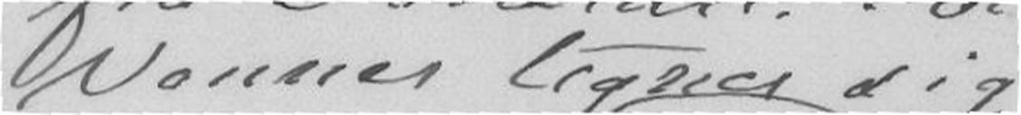Venner tegner sig
